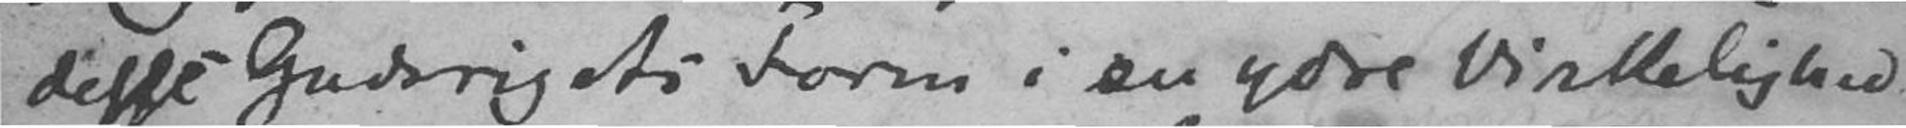delse Gudsrigets Form i en ydre Virkelighed
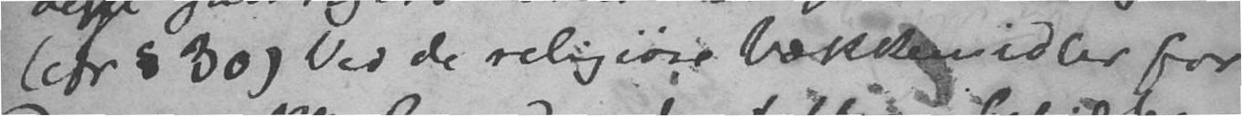(cfr § 30) Ved de religiøse Vækkemidler for
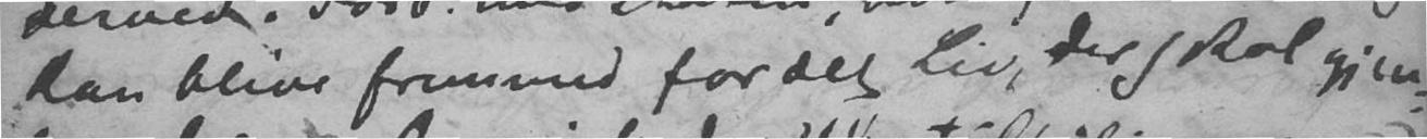kan blive fremmed for det Liv, der skal gjen-
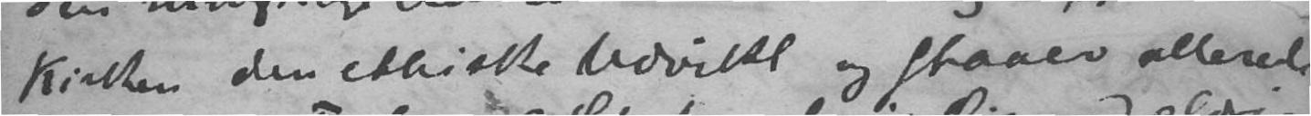Kirken den ethiske Udvikl og staaer allerede
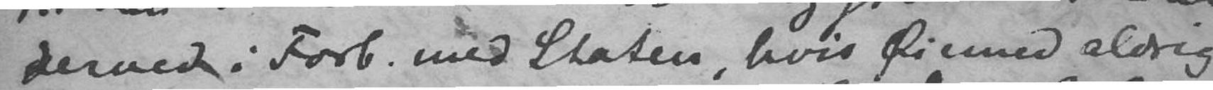derved i Forb. med Staten, hvis Øiemed aldrig
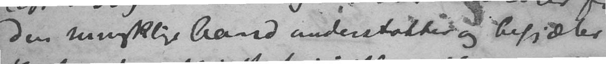den mennesklige Aand andenstalte og besjæler
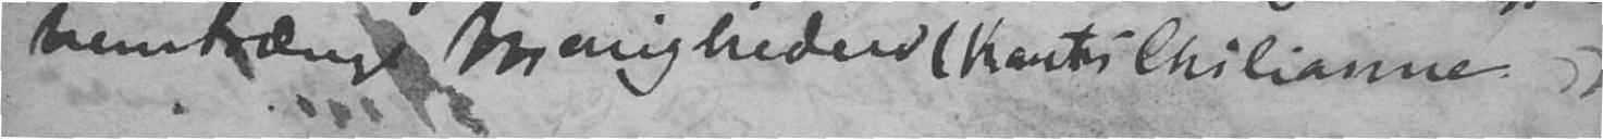nemtrænge Menigheden (Kants Chiliasme)
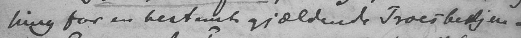ning for en bestemt gjældende Troesbekjen-
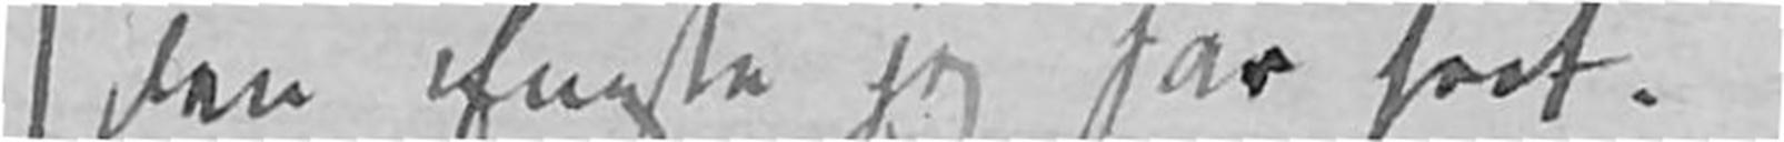den Eneste jeg har seet.
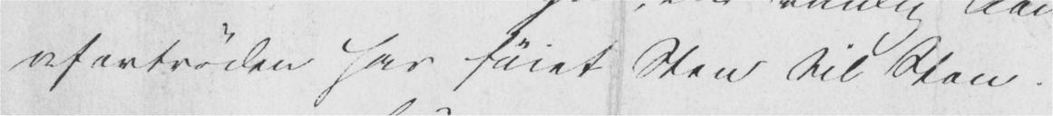ufortrøden har føiet Sten til Sten.
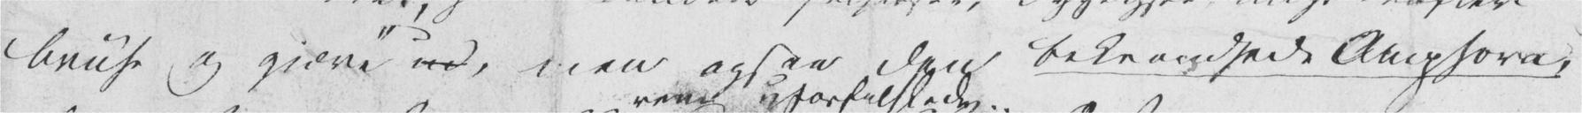bruse og gjære» ud, men ogsaa den bekrandsede Amphora,
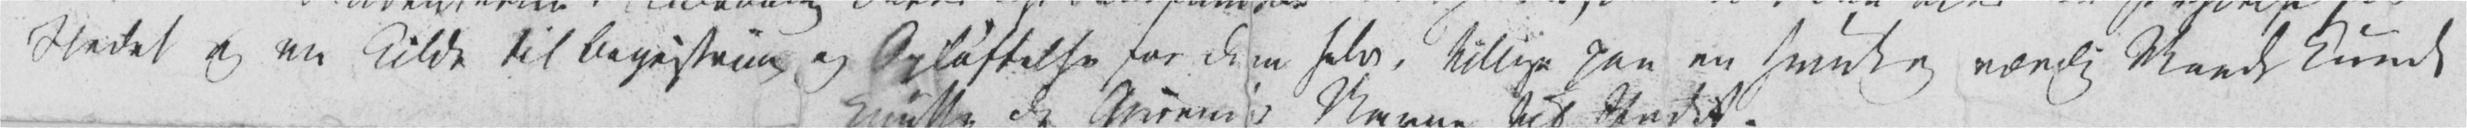Stedet og en Kilde til Begeistring og Opløftelse for dem selv, tillige paa en smuk og værdig Maade kunde
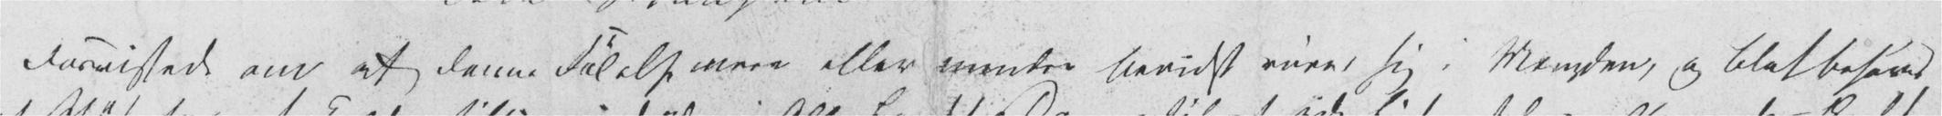Forvissede om at denne Følelse mere eller mindre bevidst rører sig i Mængden, og blot behøver
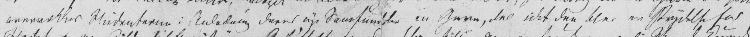overrækkes Studenterne i Anledning deres nye Samfundsb. en Gave, der idet den blev en Prydelse for
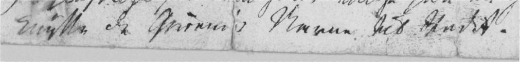knytte de Givendes Navne til Stedet.
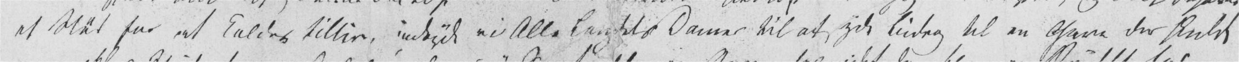et Stød for at kaldes tillive, indbyde vi Alle Landets Damer til at yde Bidrag til en Gave der skulde
